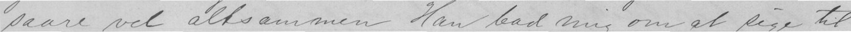saare vel altsammen. Han bad mig om at sige til
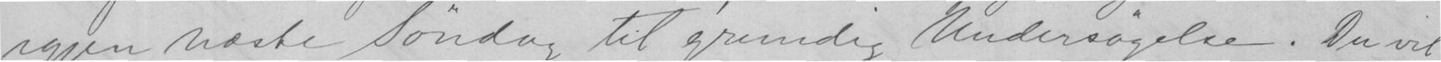igjen næste Søndag til grundig Undersøgelse. Du vil
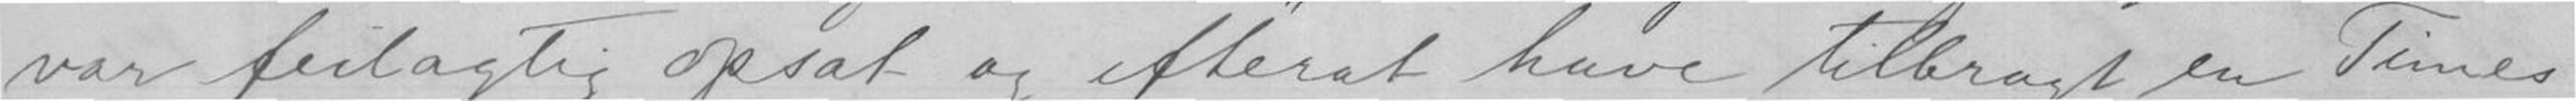var feilagtig opsat og efterat have tilbragt en Times
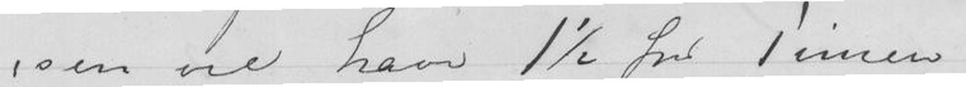sen vil have 1½ spd Timen
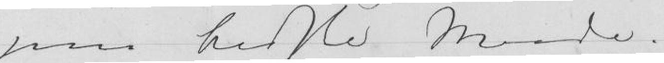paa bedste Maade.
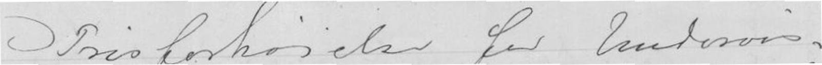Prisforhøielse for Undervis-
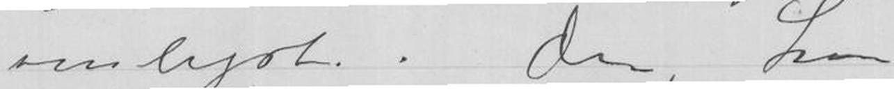venligste.Din hen.
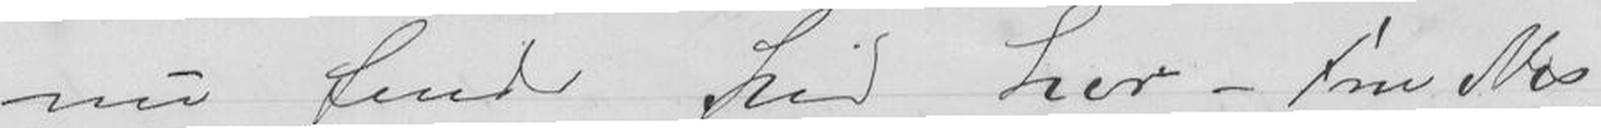nu finde hid her - Fru Nis-
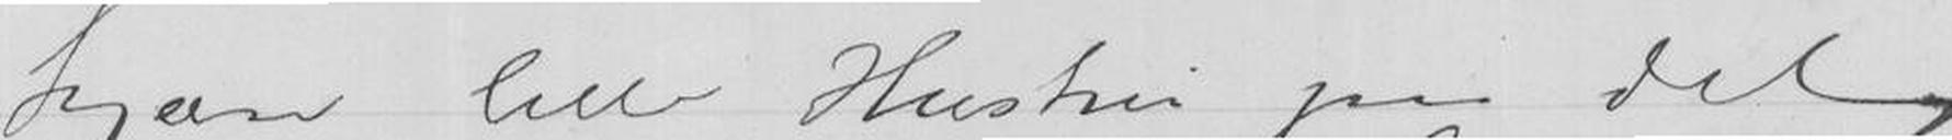kjære lille Hustru paa det
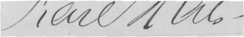Karl Hals
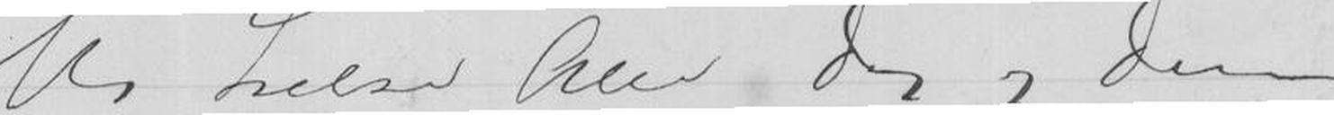Vi hilse Alle Dig og Din
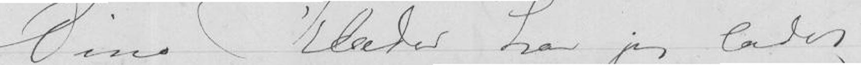Dine Klæder har jeg ladet
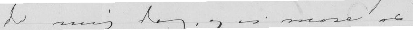der mig dog, og vi more os
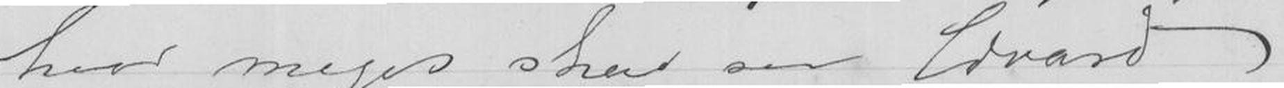hvor meget skal saa Edvard
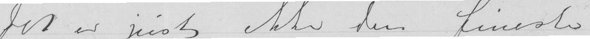det er just ikke den fineste
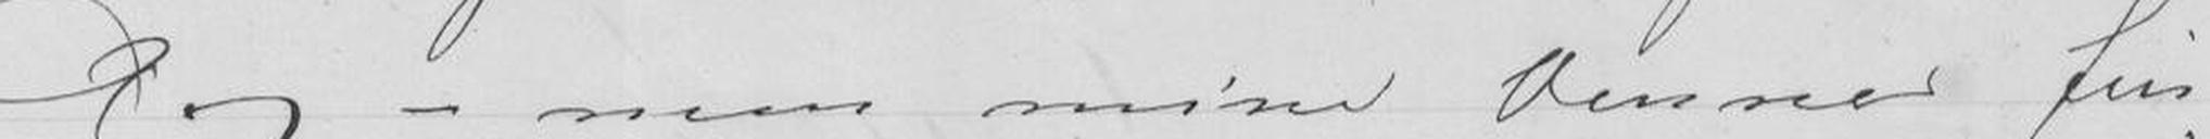Dag - men mine Venner fin-
